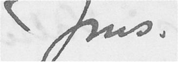Jens.
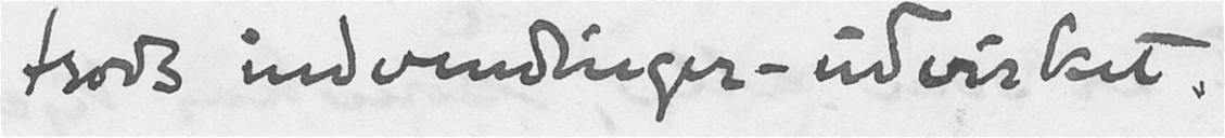trods indvendinger - udvirket.
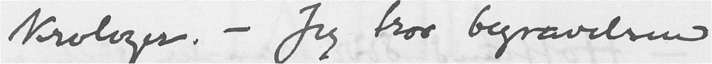krologer. - Jeg tror begravelsen
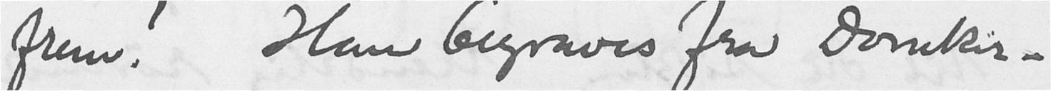frem! Han begraves fra Domkir-
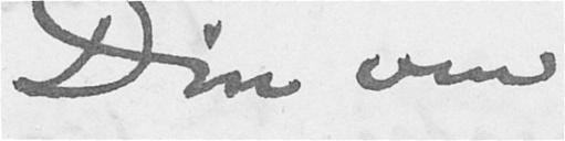Din ven
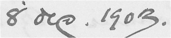8 dec. 1903.
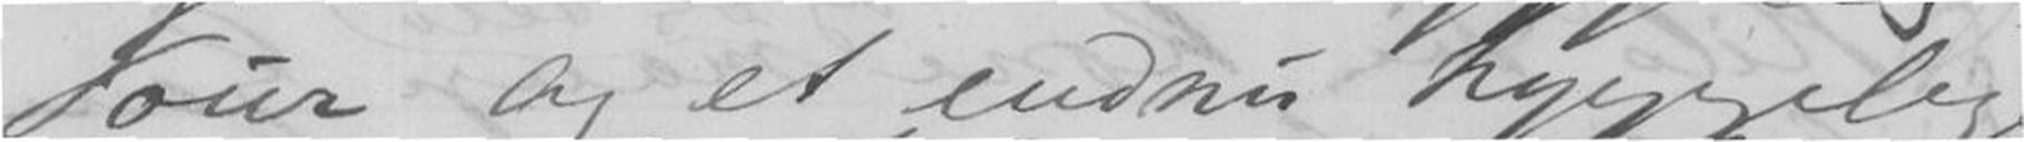Tour og et endnu hyggelig
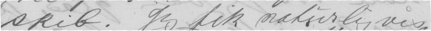skib. Jeg fik naturligvis
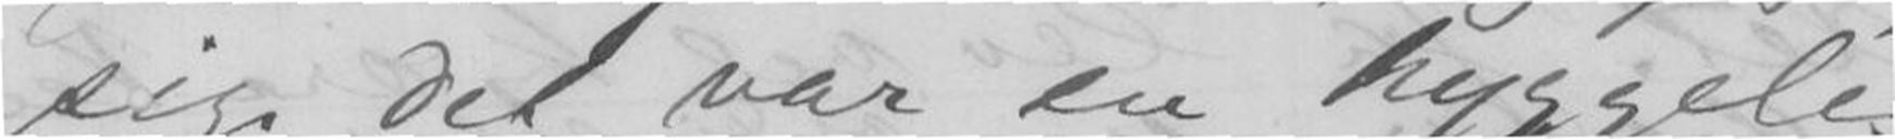sige det var en hyggelig
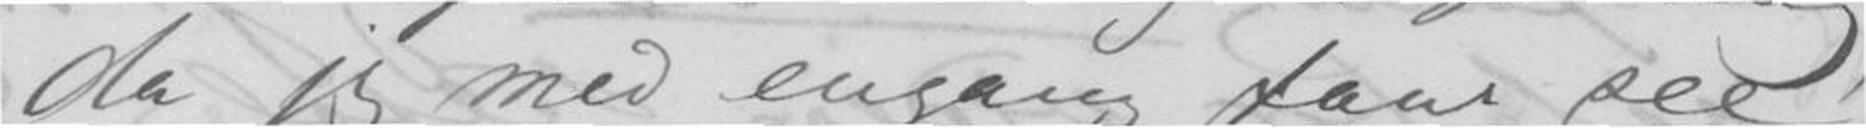da jeg med engang faar see
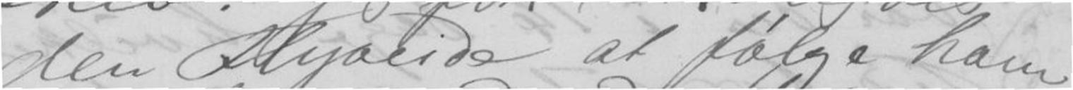den Flyaeide at følge ham
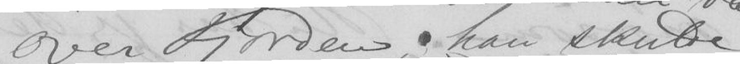over Fjorden; han skulde
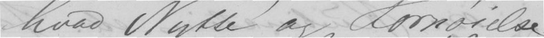hvad Nytte og Fornøielse
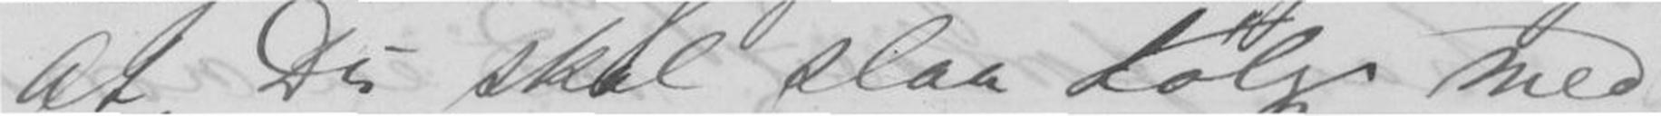At Du skal følge med
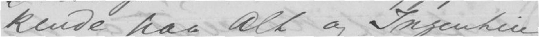kende paa Alt og Ingenting,
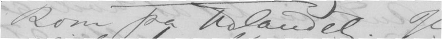kom fra Udlandet. Jeg
